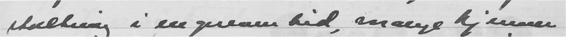staltning i en opreven tid, mange kjenner
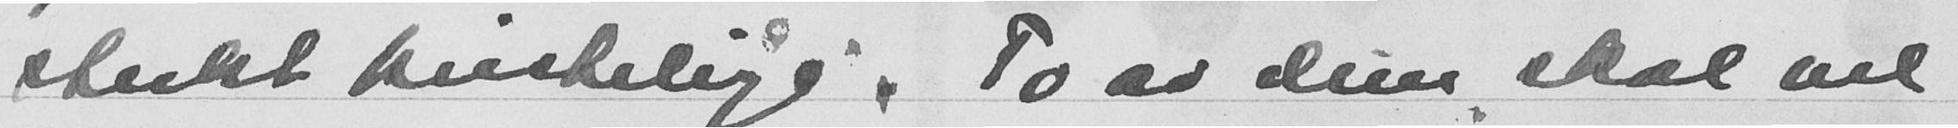sterkt kristelige. To av dem, skal vel
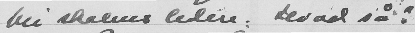bli skolens ledere. Hvad så?
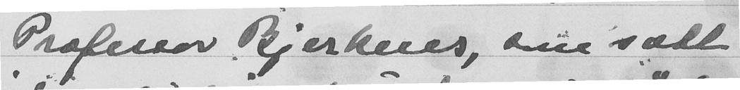Professor Bjerknes, som satt
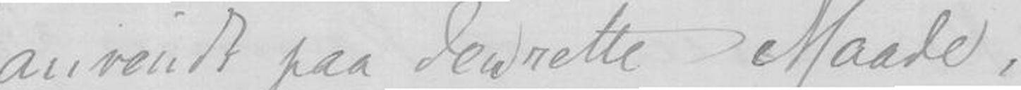anvendt paa den rette Maade,
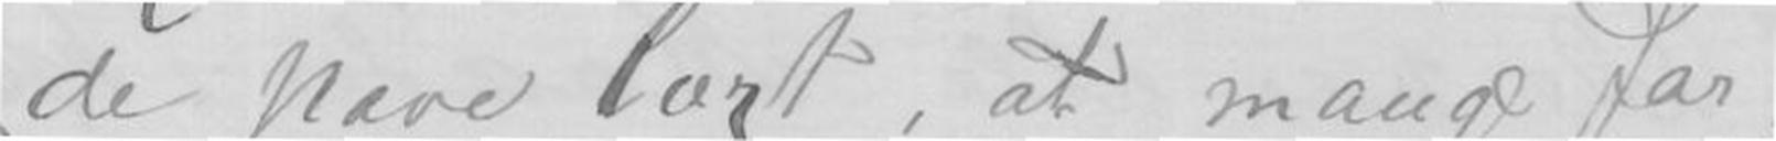de spare lort , at mange for
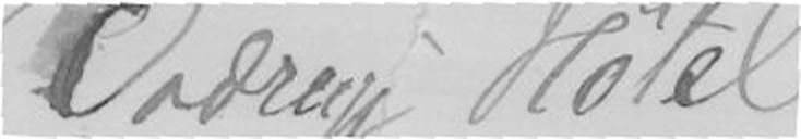Dadrup Hotel
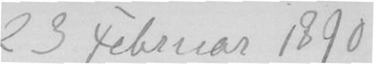23 Februar 1890
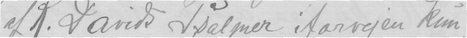af K. Davids Psalmer i forvejen kun
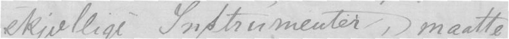skjællige Instrumenter, maatte
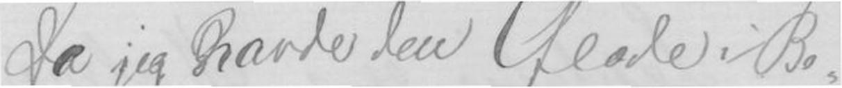Da jeg havde den Glæde i Be-
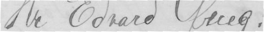Hr. Edvard Grieg !
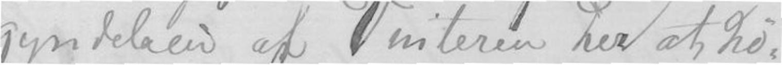gundelsen af Vinteren her at hø-
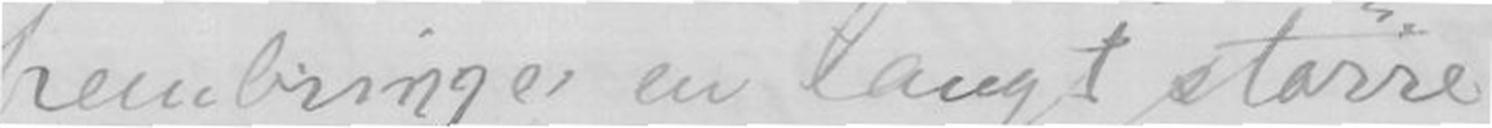frembringe en langt større
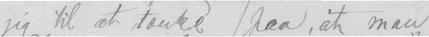jeg til at tænke paa, at man
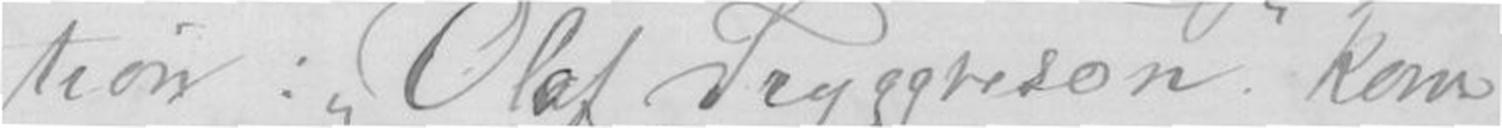tion: Olaf Tryggvason kom
